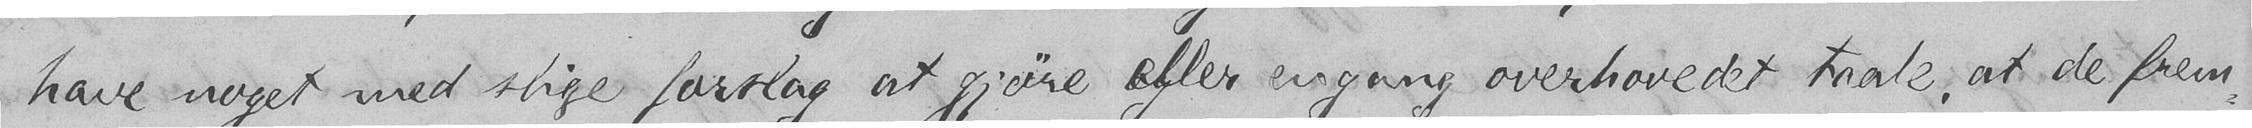have noget med slige forslag at gjøre eller engang overhovedet taale, at de frem-
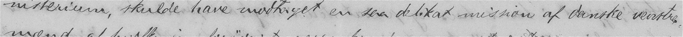nisterium, skulde have modtaget en saa delikat mission af danske venstre-
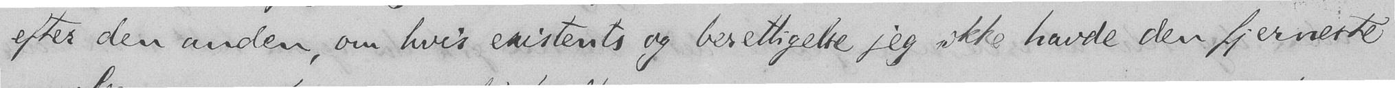efter den anden, om hvis existents og berettigelse jeg ikke havde den fjerneste
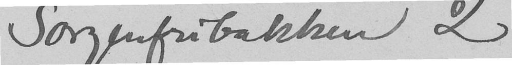Sorgenfribakken 2
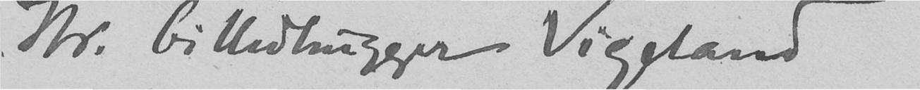Hr. billedhugger Vigeland
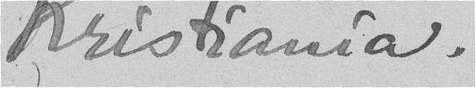Kristiania.
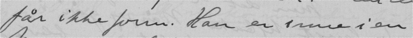får ikke form. Han er inne i en
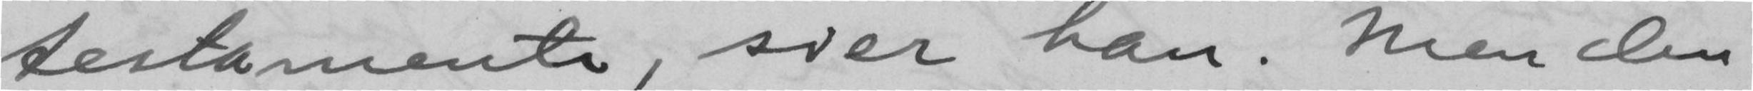testamente, sier han. Men den
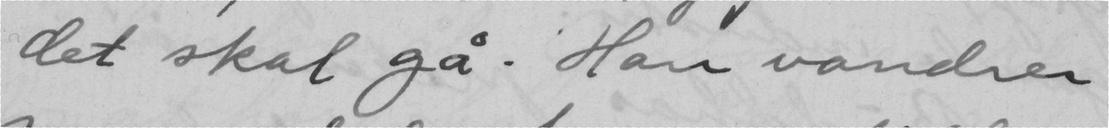det skal gå. Han vandrer
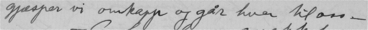gjæsper vi omkapp og går hver til oss -
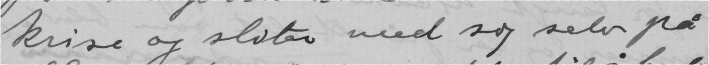krise og sliter med sig selv på
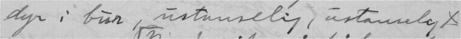dyr i bur, ustanselig, ustanselig x
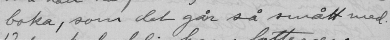boka, som det går så smått med.
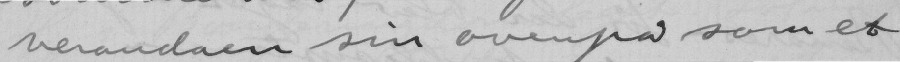verandaen sin ovenpå som et
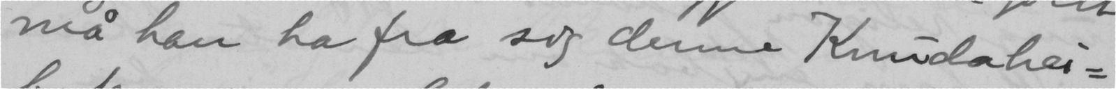må han ha fra sig denne Knudahei-
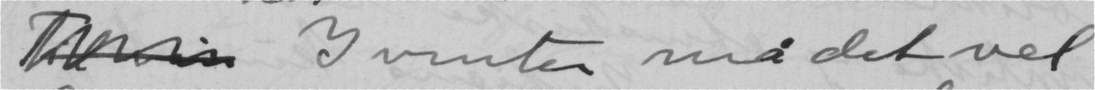I vinter må det vel
